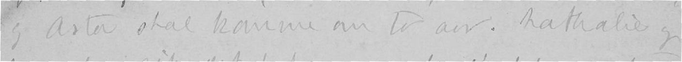og Asta skal komme om to aar. Nathalie og
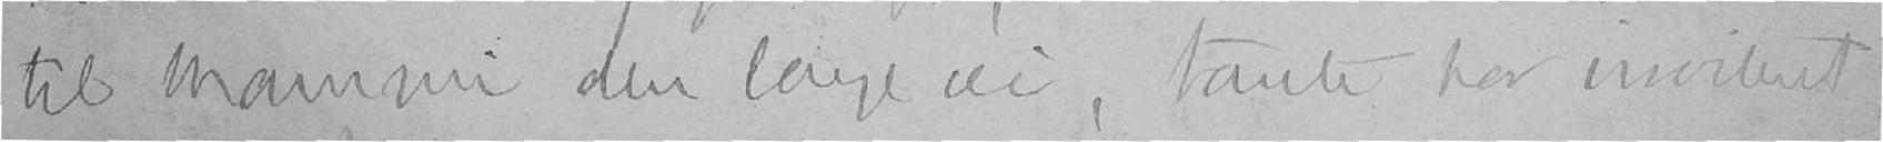til Mammi den lange vei, tante har inviteret
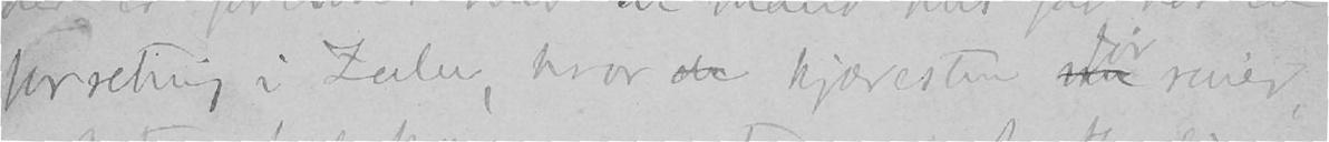forretning i Zulu, hvor en kjæresten nu før reiser,
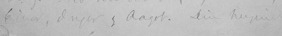Einar, Ingar og Aagot. Din hengivne
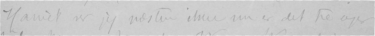Harriet ser jeg næsten ikke nu er det tre uger
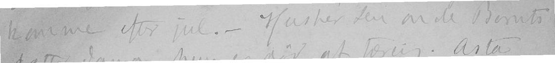komme efter jul. – Husker Du oncle Bernts
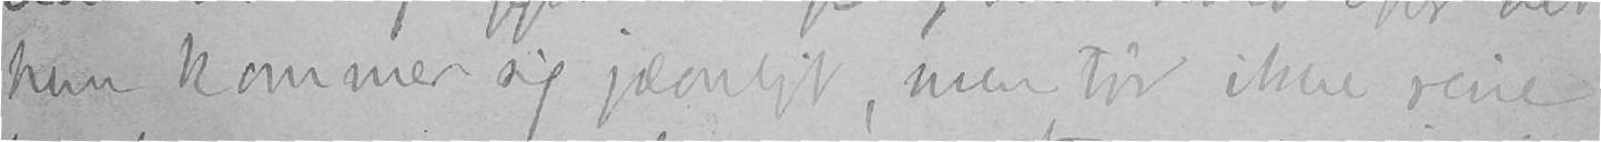hun kommer sig jævnligt, men tør ikke reise
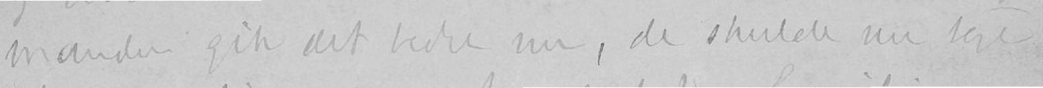manden gik det bedre nu, de skulde nu tage
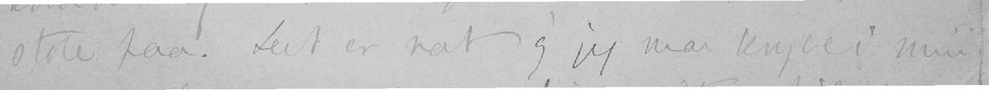stole paa. Det er nat og jeg maa krybe i min
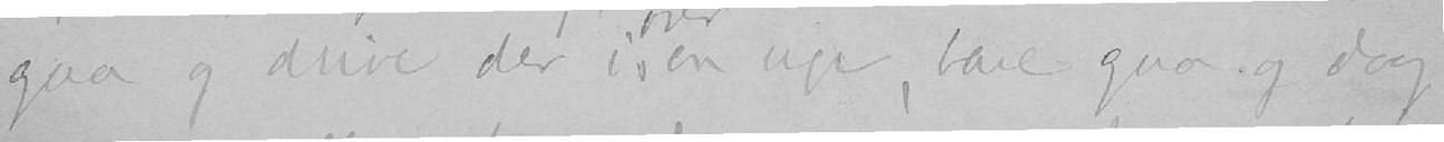gaa og drive der i en uge, bare gaa og dag-
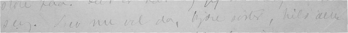seng. Lev nu vel da, kjære søster, hils alle
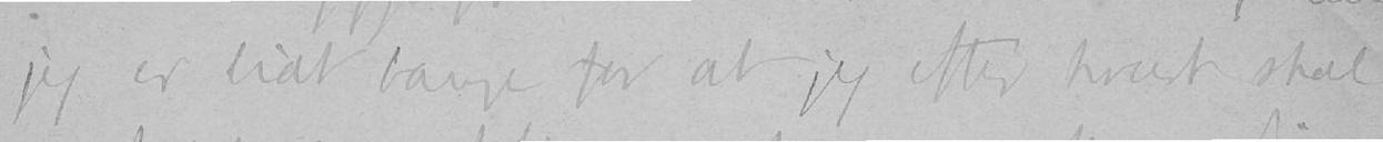jeg er lidt bange for at jeg efter hvert skal
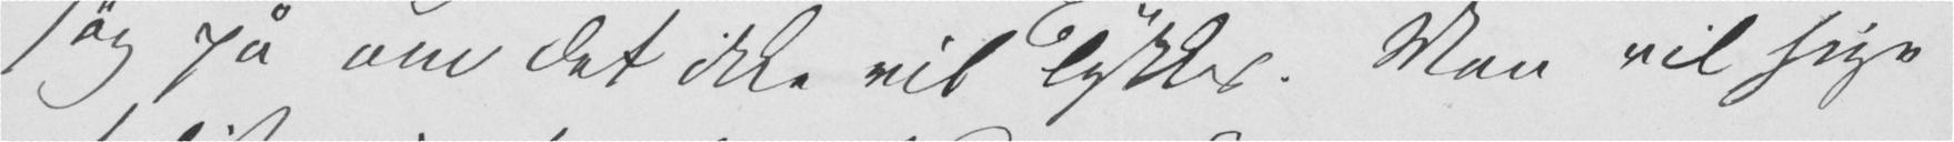søg på om det ikke vil lykkes. Man vil sige
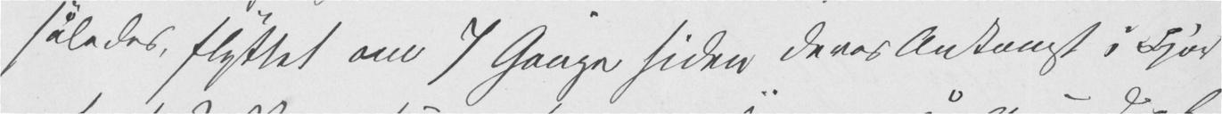således, flyttet om 7 Gange siden deres Ankomst i Fjor
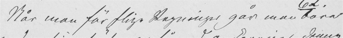Når man får slige Regninger går man bare
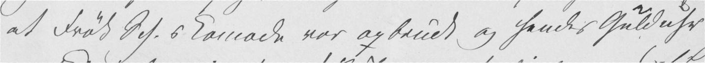at Frøk Sch.s Komode var opbrudt og hendes Gulduhr
